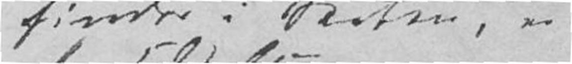findes i Staten, er
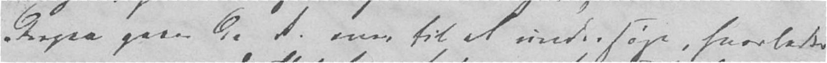Derpaa gaaer da A. over til at undersøge, hvorledes
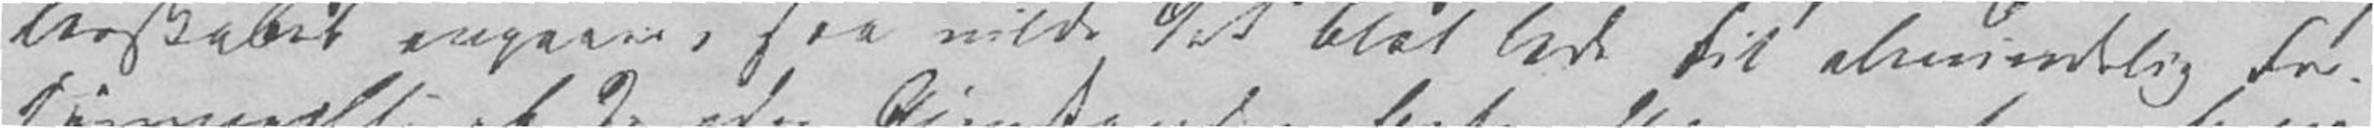lesskabet angaaer, saa vilde det blot lede til almindelig For-
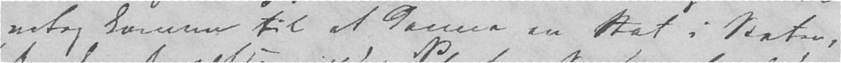netop komme til at danne en Stat i Staten,
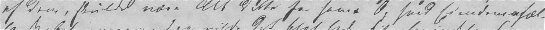af dem, skulde være Alt dette for ham. Og hvad Eiendomsfæl-
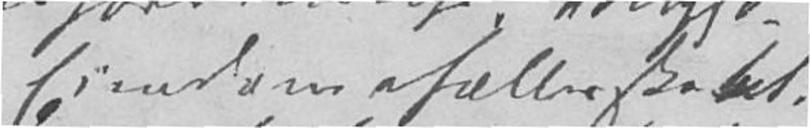Eiendomsfælleskabet.
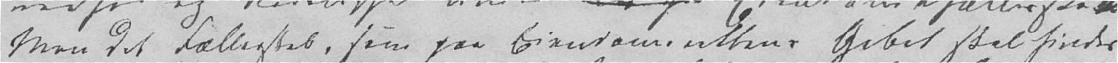Men det Fællesskab, som paa Eiendomsrettens Gebet skal findes
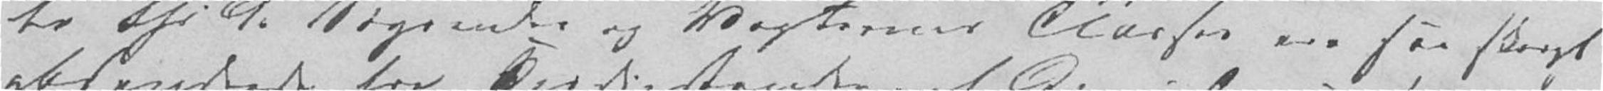to thi de Styrendes og Vogternes Classer ere saa skarpt
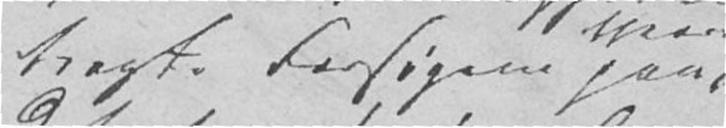tragte Forsøgene paa
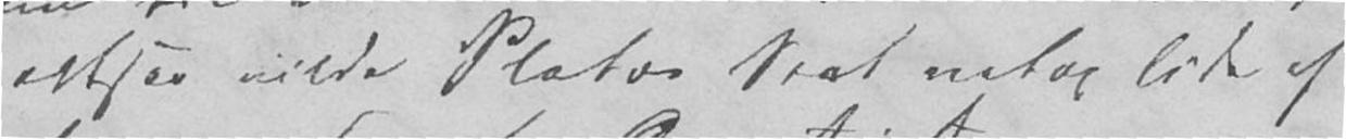altsaa vilde Platos Stat netop lide af
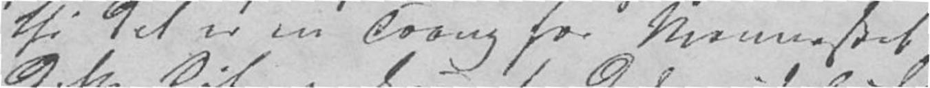thi det er en Trang for Mennesket
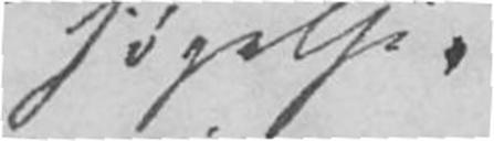søgelse.
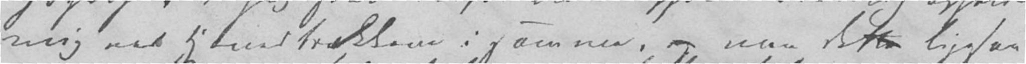mig ved Hovedtrækkene i samme, og men dette ligesaa
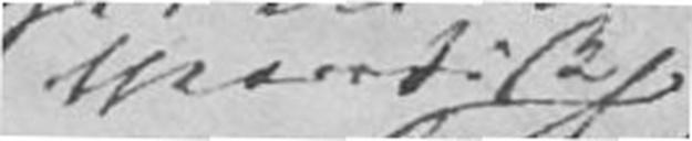theoretisk
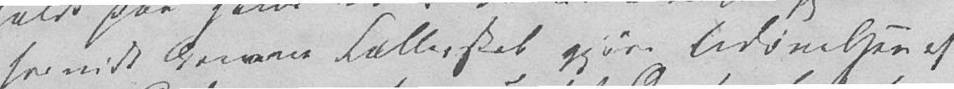for vidt drevne Fællesskab gjøre Udøvelsen af
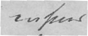enhver
